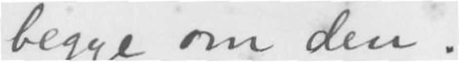begge om den.-
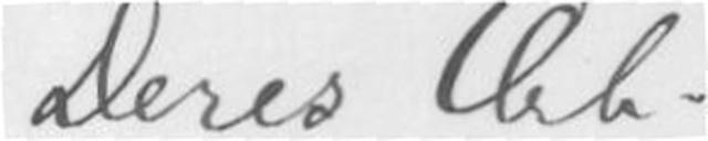Deres Ærb.
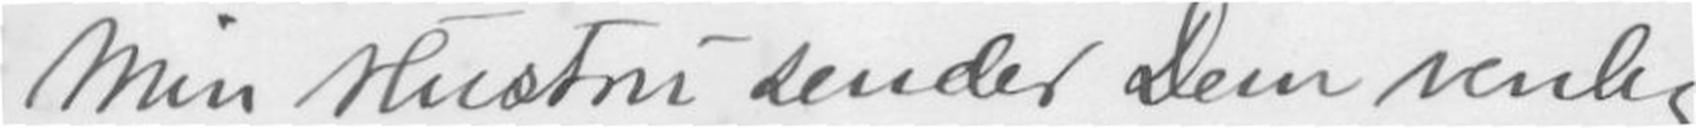Min Hustru sender Dem venlig
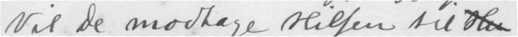Vil De modtage Hilsen til Hu
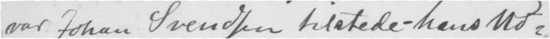var Johan Svendsen tilstede-hans Ud?
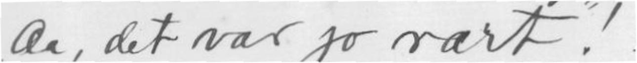Aa det var jo rart!-
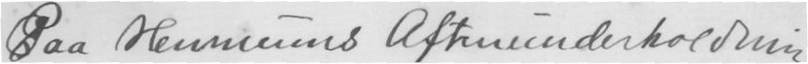Paa Hennums Aftenunderholdning
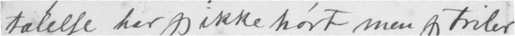talelse har jeg ikke hørt men jeg tviler
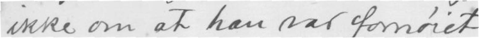ikke om at han var fornøiet.
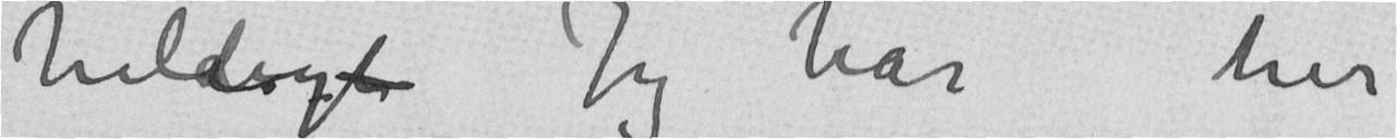heldigtJeg har her
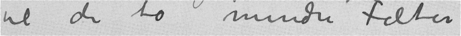til de to mindre Fælter
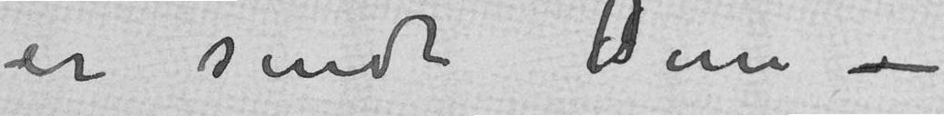er sendt Dem –
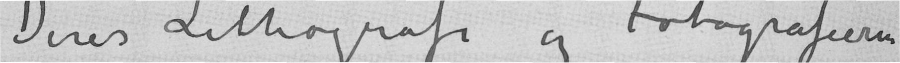Deres Lithografi og Fotografierne
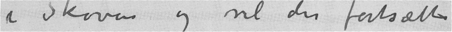i Skoven og vil der fortsætte
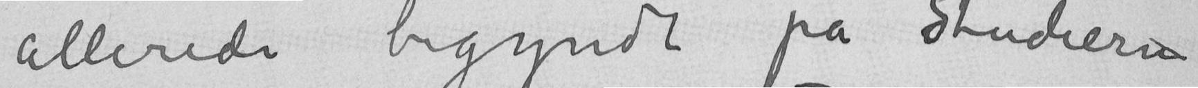allerede begyndt på Studierne
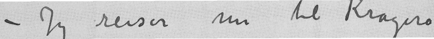– Jeg reiser nu til Kragerø
